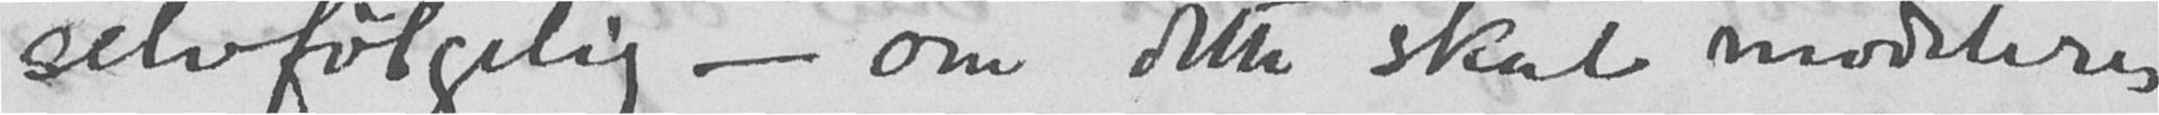selvfølgelig - om dette skal modeleres
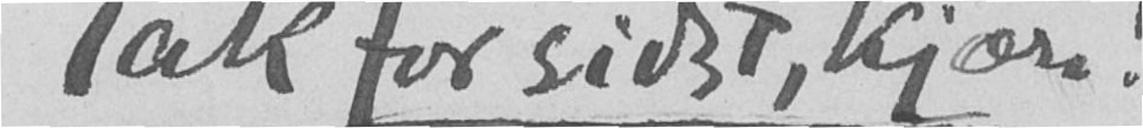Takk for sidst, kjære!
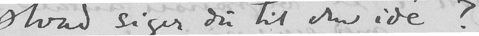Hvad siger du til den ide?
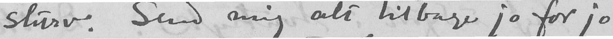slurv. Send mig alt tilbage jo før jo
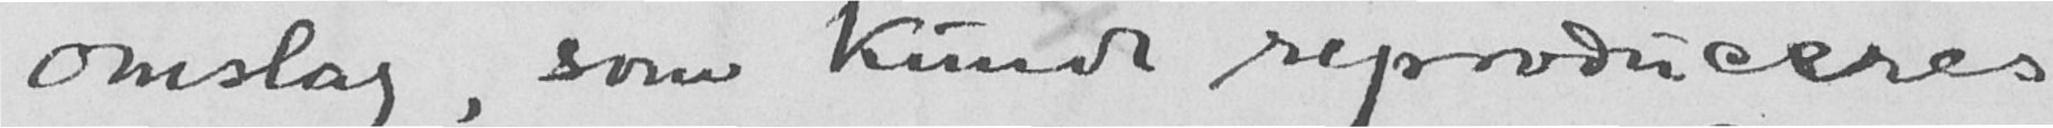omslag, som kunde reproduceres
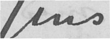Jens
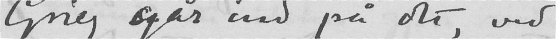Grieg går ind på det, ved
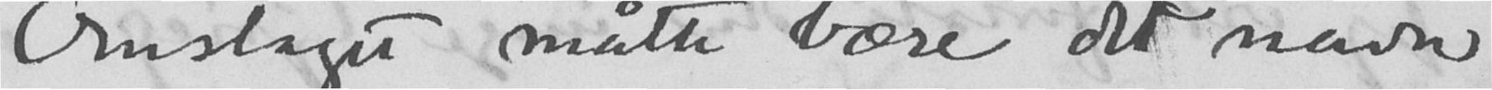Omslaget måtte bære dit navn
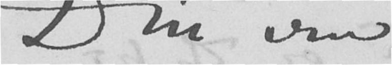Din ven
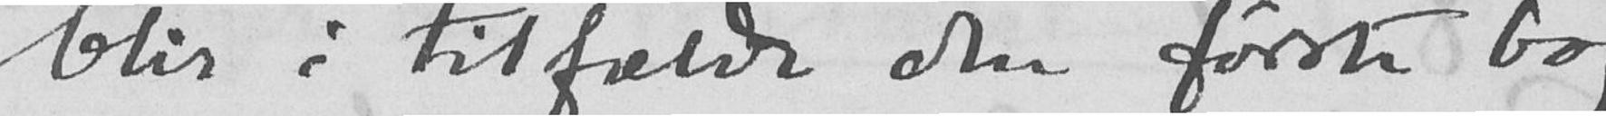blir i tilfælde den første bog
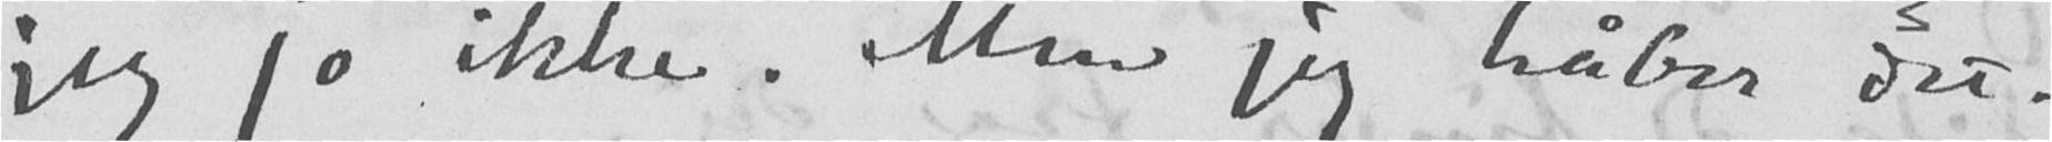jeg jo ikke. Men jeg håber det.
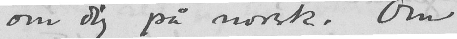om dig på norsk. Om
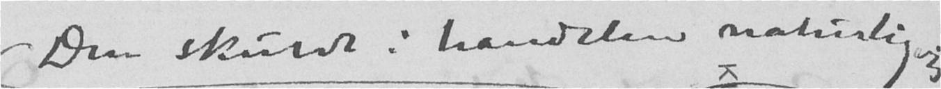Den skulde i handelen naturligvis
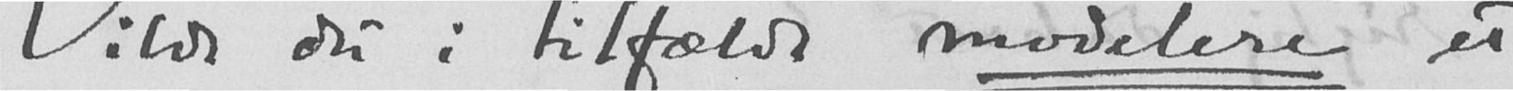Vilde du i tilfælde modelere et
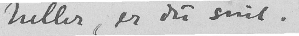heller, er du snil.
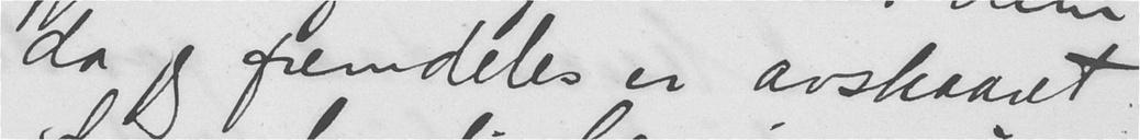da jeg fremdeles er avskaaret
